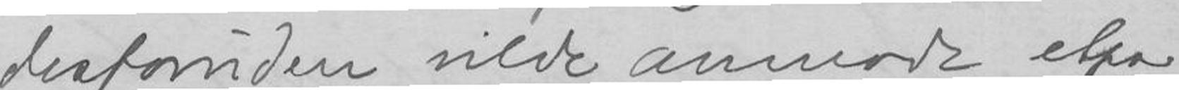desforuden vilde anmode etpar
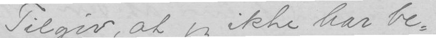Tilgiv, at jeg ikke har be-
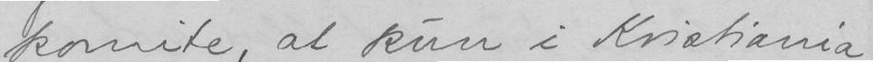komite, at kun i Kristiania
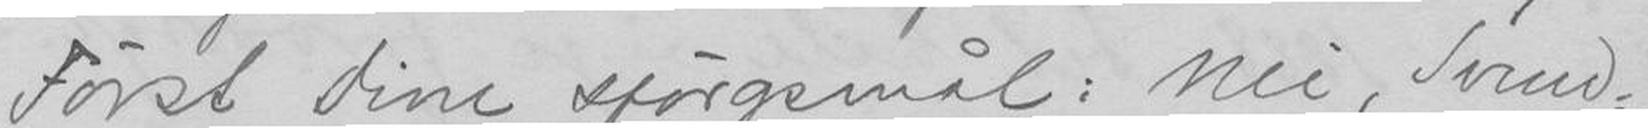Først dine spørgsmål: Nei, Svend-
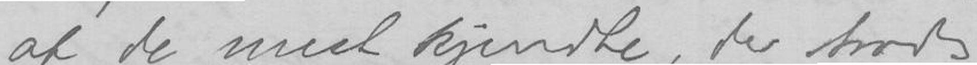af de mest kjendte, der trods
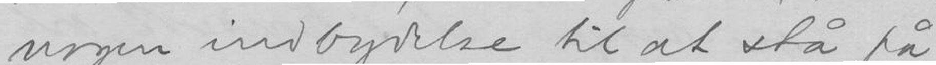nogen indbydelse til at stå på
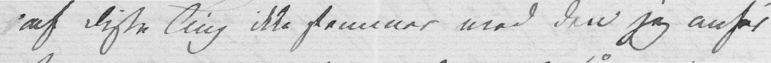af disse Ting ikke stemmer med den jeg anser
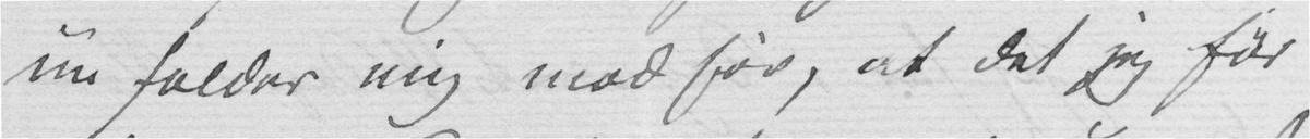nu falder mig mod før, at det jeg før
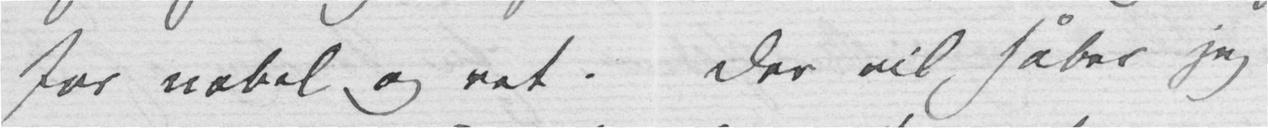for nobel og ret. Der vil håber jeg
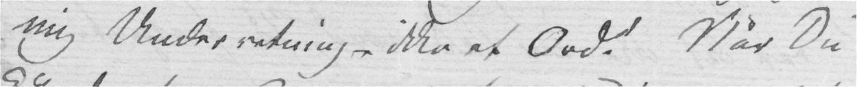mig Underretning – ikke et Ord. Når Du
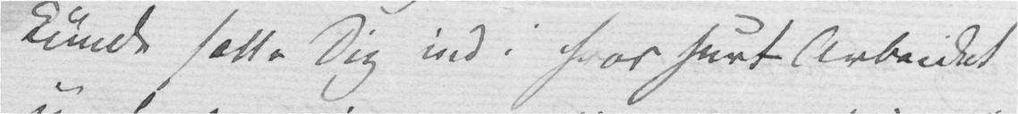kunde sætte Dig ind i hvor surt Arbeidet
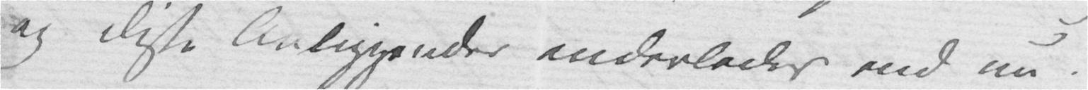og disse Anliggender anderledes end nu.
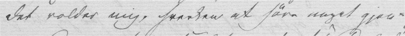det volder mig, hverken at høre noget gjen-
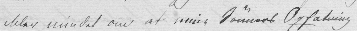blev mindet om at mine Sønners Opfatning
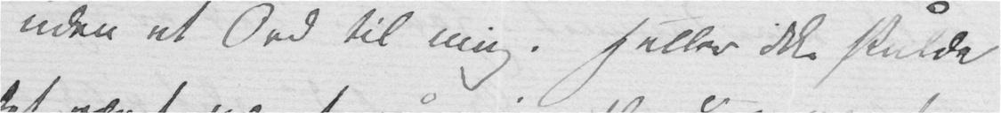uden et Ord til mig. Heller ikke skulde
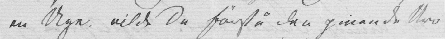en Uge, vilde Du forstå den pinende Uro
